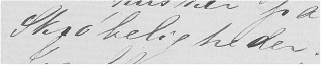Skrøbeligheder.
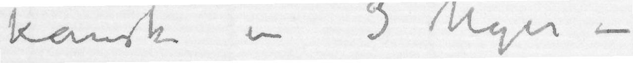kanskje end 3 Uger –
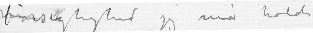Forsigtighed jeg må holde
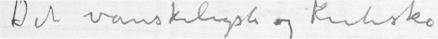Det vanskeligste og Kritiske
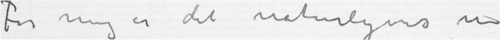For mig er det naturligvis nu
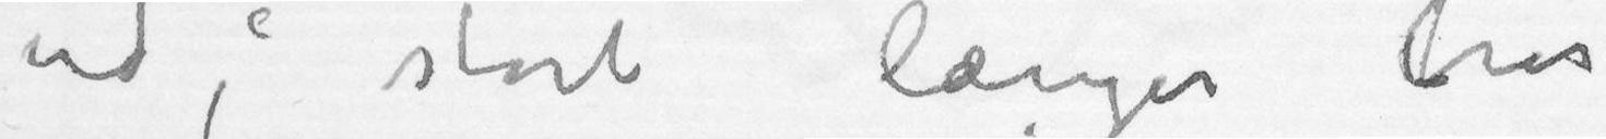ud; stort længer tror
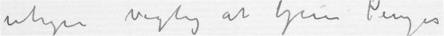uhyre vigtig at tjene Penger
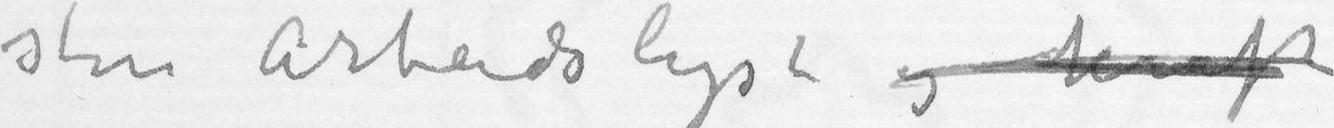store Arbeidslyst og kraft
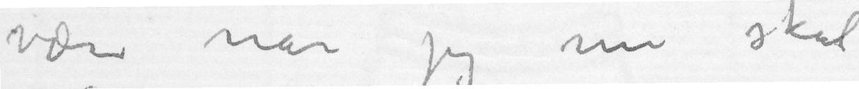være når jeg nu skal
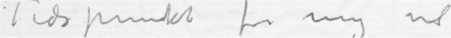Tidspunkt for mig vil
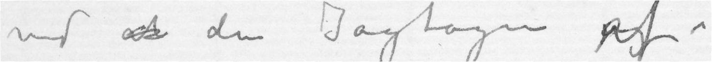ved at den Iagtagen af-
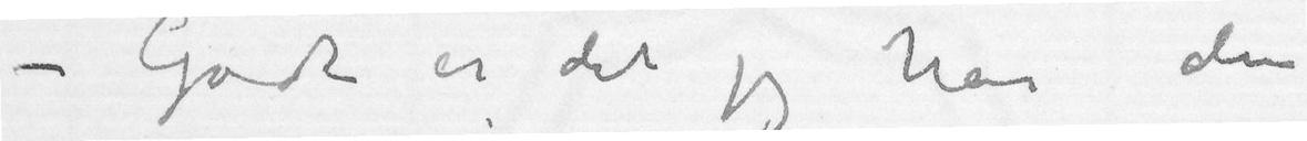– Godt er det jeg har den
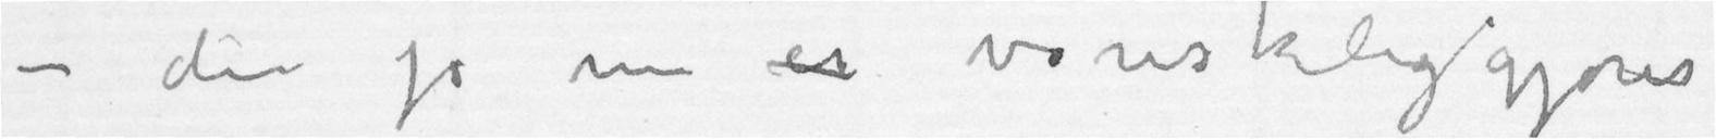– der jo nu er vanskeliggjøres
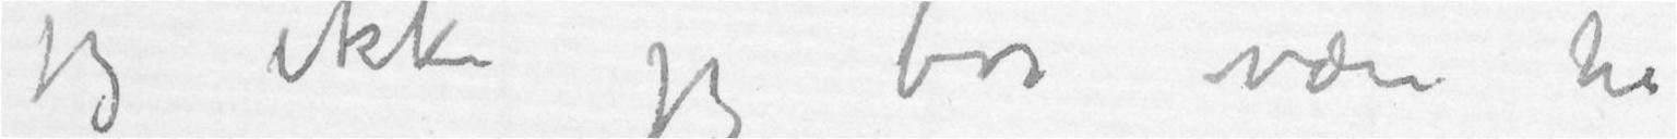jeg ikke jeg bør være her
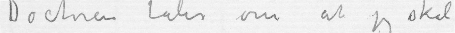Doctoren taler om at jeg skal
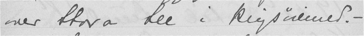over Stora Lee i krigsøiemed. -
